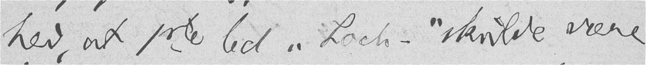hed, at 1ste led "Loch-" skulde være
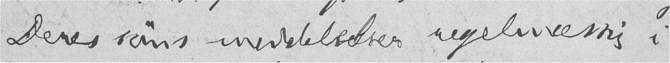Deres søns meddelelser regelmæssig i
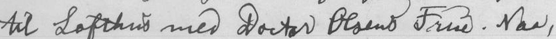til Lofthus med Doctor Olsens Frue. Naa,
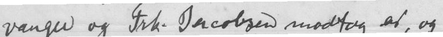vanger og Frk. Jacobsen modtog os, og
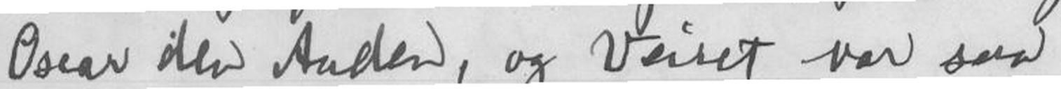Oscar den Anden, og Veiret var saa
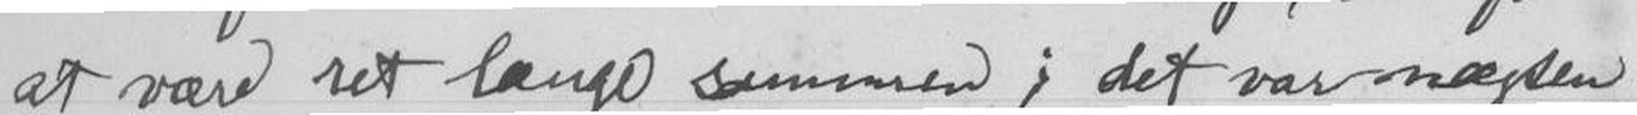at være ret længe sammen; det var næsten
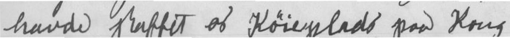havde skaffet os Køieplads paa Kong
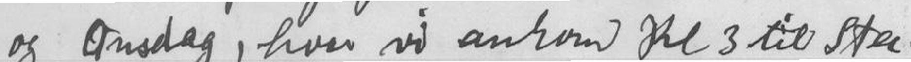og Onsdag, hvor vi ankom Kl 3 til Sta-
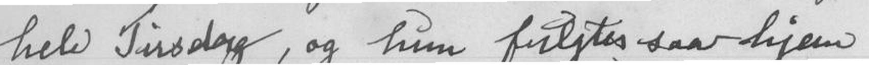hele Tirsdag, og hun fulgtes saa hjem
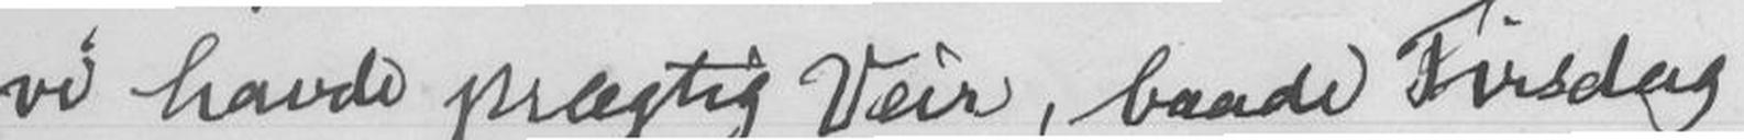vi havde prægtig Veir, baade Tirsdag
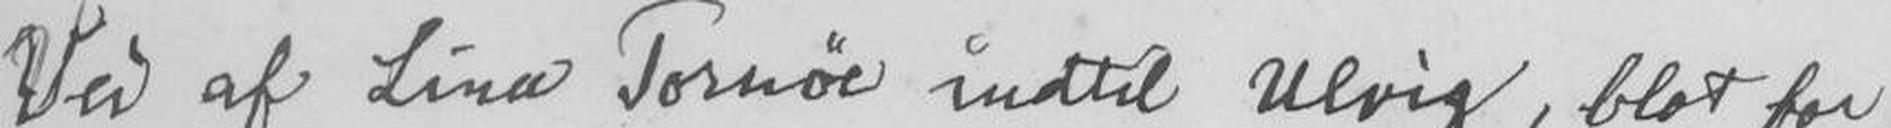Vei af Lina Tornøe indtil Ulvig, blot for
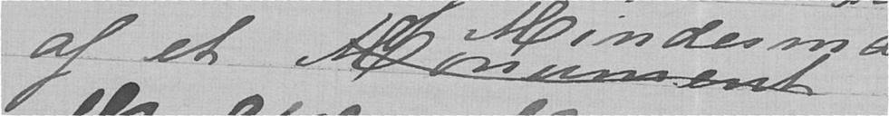af et Monument
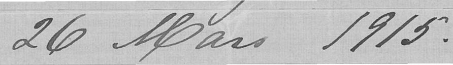26 Mars 1915.
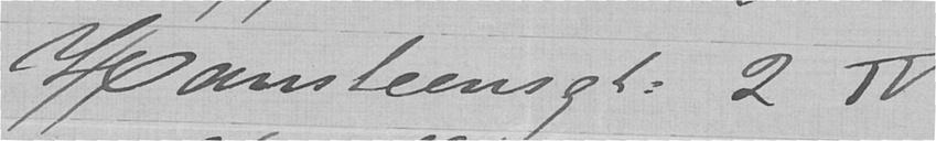Hansteensgt. 2 IV
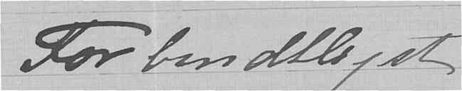Forbindtligst
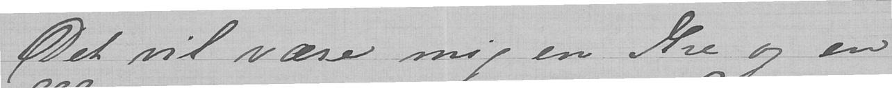Det vil være mig en Ære og en
